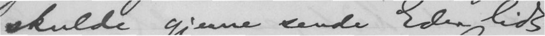skulde gjerne sende Eder lidt
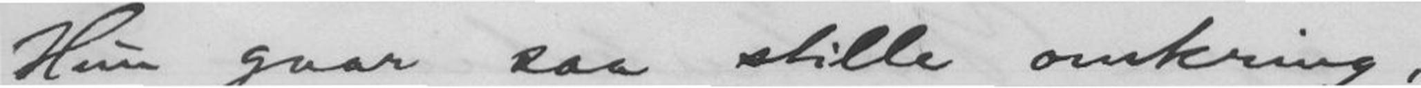Hun gaar saa stille omkring,
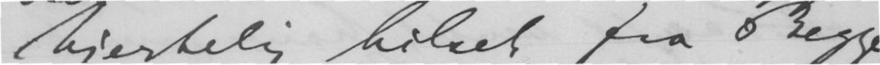hjertelig hilset fra Begge!
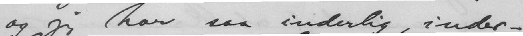og jeg har saa inderlig, inder-
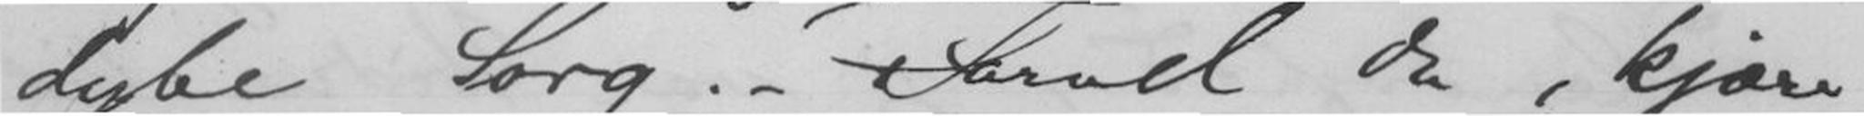dybe Sorg. - Farvel da, kjære
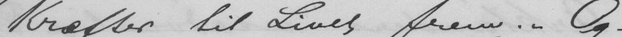Kræfter til Livet frem. Og-
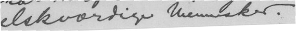elskværdige Mennesker.
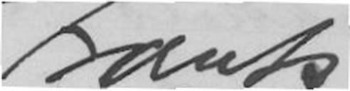Frants.
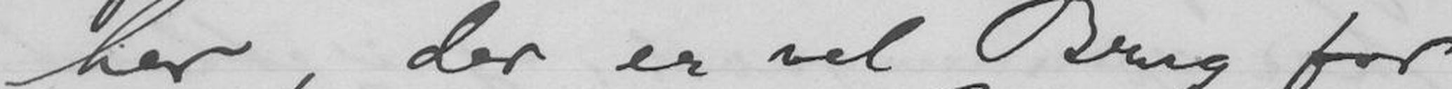her, der er vel Brug for
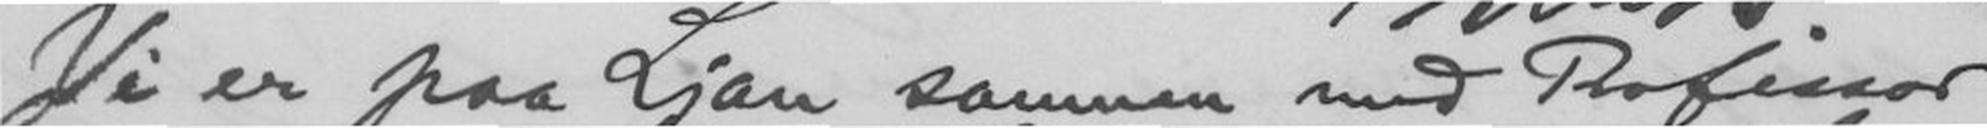Vi er paa Ljan sammen med Professor
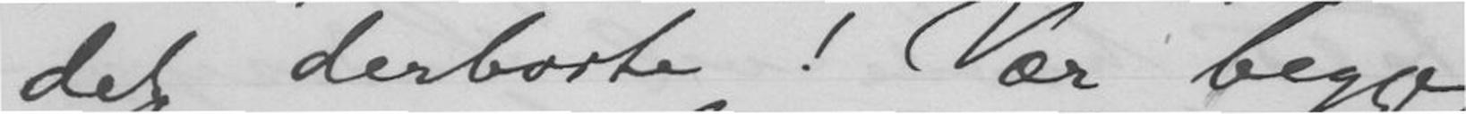det derborte! Vær begge
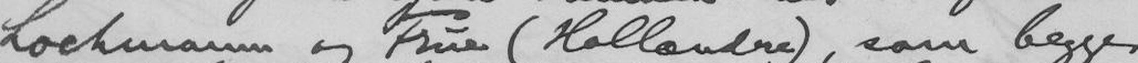Lochmann og Frue (Hollændre), som begge
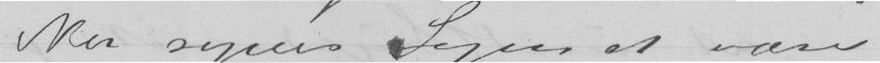Nu synes Sagen at være
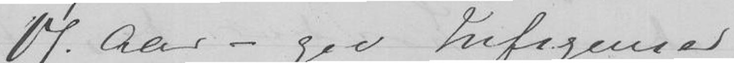17 Aar - gav Infigenia
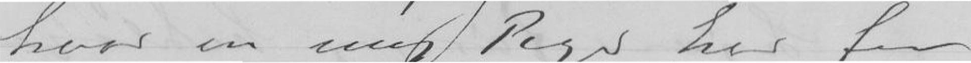hvor en ung Pige her fra
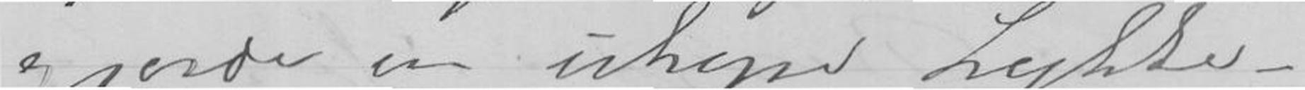gjorde en uhyre Lykke -
